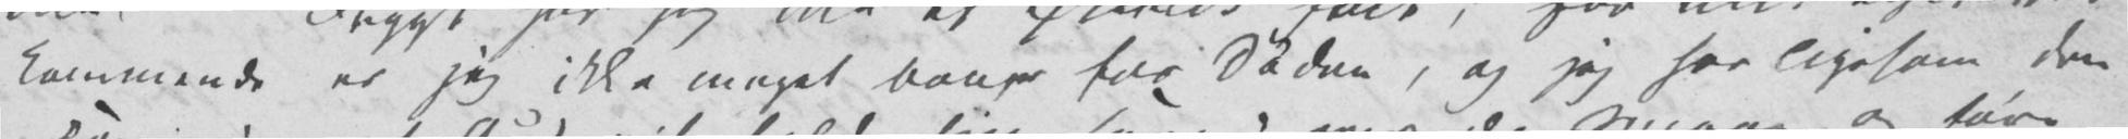kommende er jeg ikke meget bange for Døden, og jeg har ligesom den
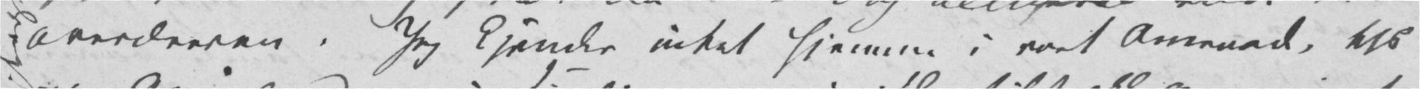overdreven. Jeg kjender intet hjemme i vort Omraade, thi
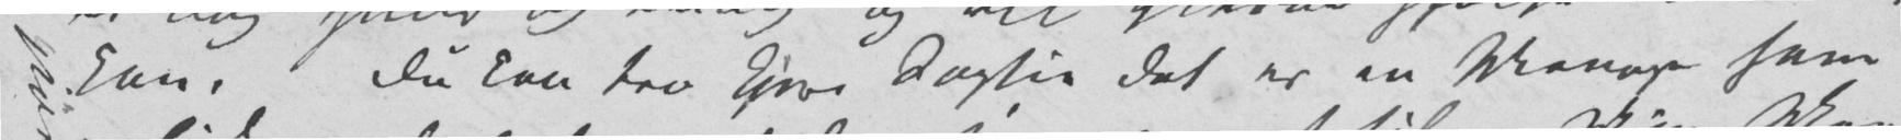kan, Du kan tro kjere Sophie det er en Menage som
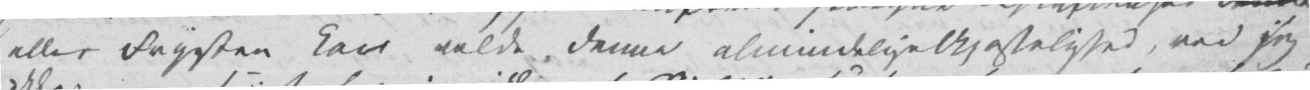eller Frygten kan volde denne almindelige Upasselighed, ved jeg
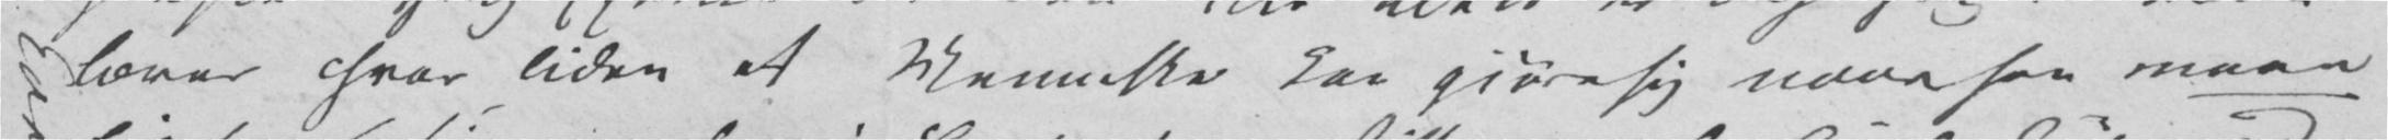lærer hvor liden et Menneske kan gjøre sig naar han maae
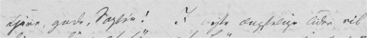Kjere, gode, Sophie! I disse ængstelige Tider vil
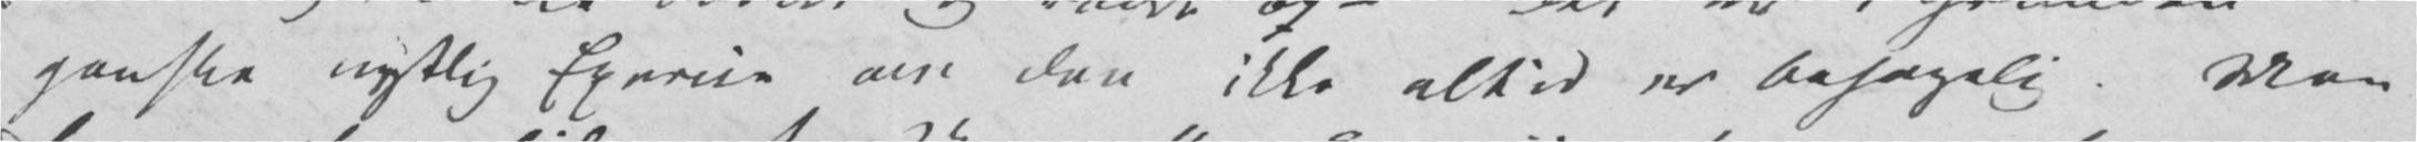ganske nyttig Exercie om den ikke altid er behagelig. Man
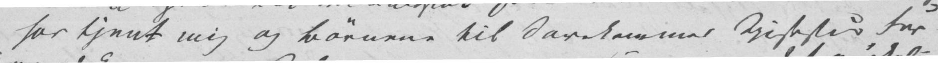har tjent mig og Børnene til Sovekammer Spisestue For-
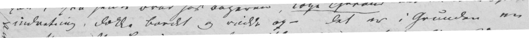indretning, dække Bordet og rødde op – det er i Grunden en
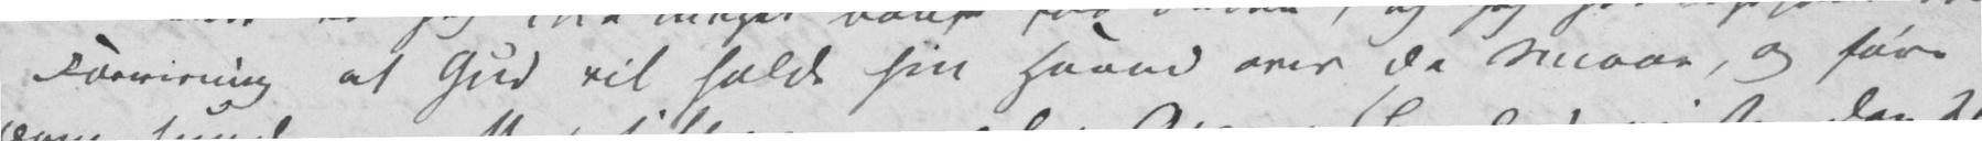Forvisning at Gud vil holde sin Haand over de Smaae, og føre
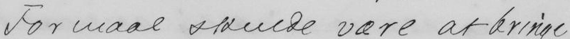Formaal skulde være at bringe
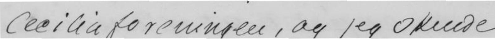Ceciliaforeningen, og jeg skulde
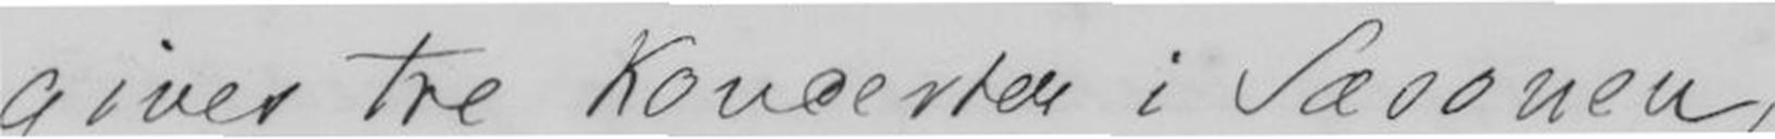gives tre Koncerter i Sæsonen,
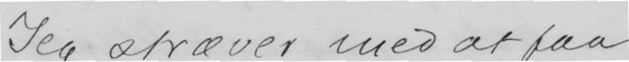Jeg stræver med at faa
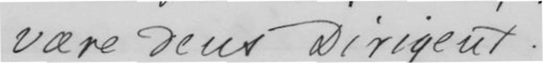være dens Dirigent.
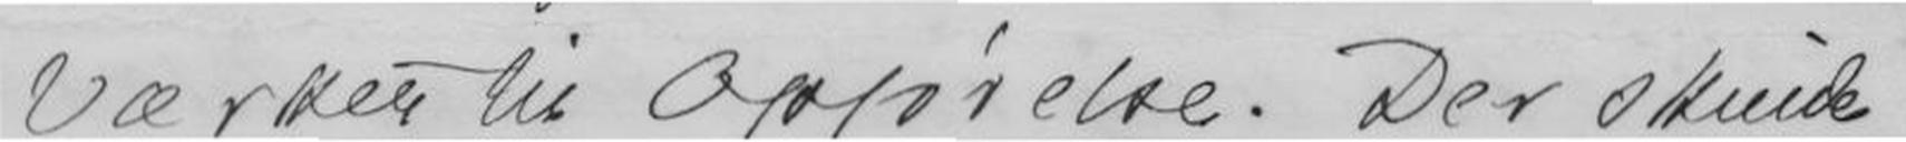værker til Opførelse. Der skulde
